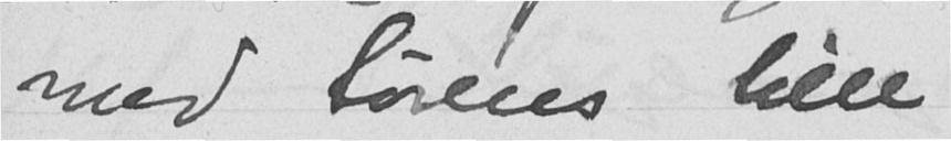med Sørens lille
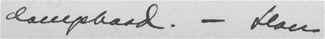dampbaad. - Han
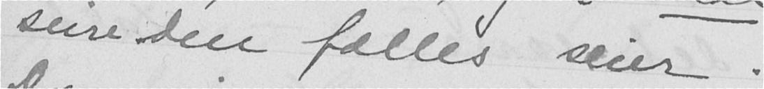seire den fælles seier.
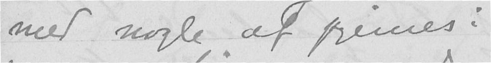med nogle af pigernes i
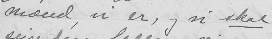mænd, vi er, og vi skal
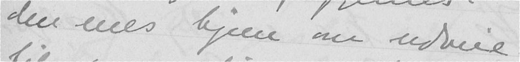den enes hjem om udveie
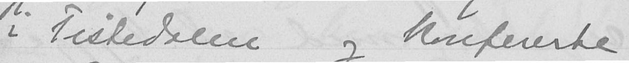i Tistedalen og konfererte
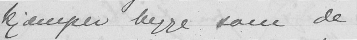kjæmper begge som de
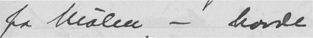fra Mølen - havde
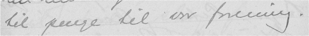til penge til vor forening.
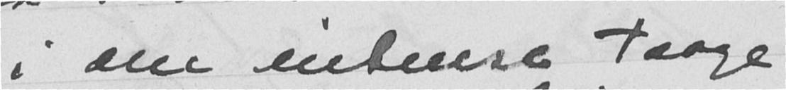i den intense taage
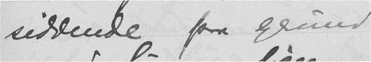siddende paa grund
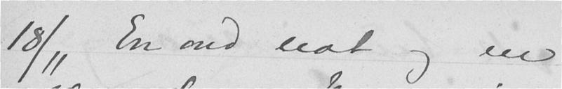18/11 En ond nat og en
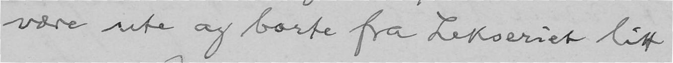være ute og borte fra Lekseriet litt
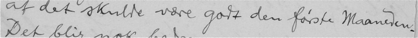at det skulde være godt den første Maaneden.
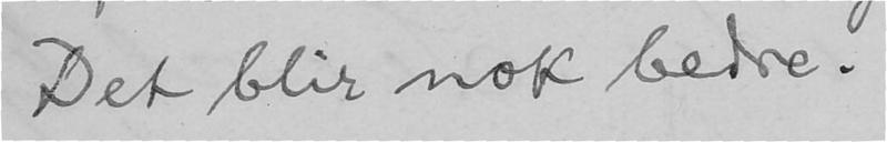Det blir nok bedre.
